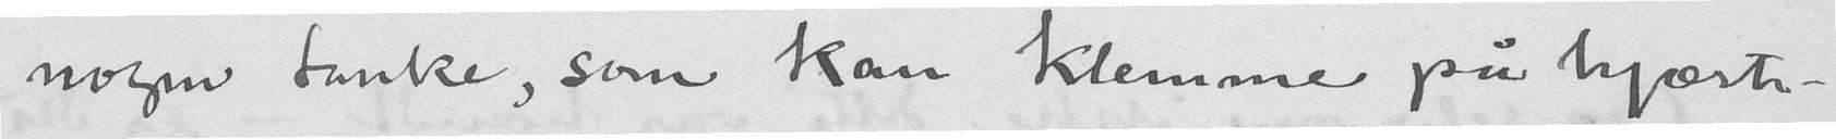nogen tanke, som kan klemme på hjærte-
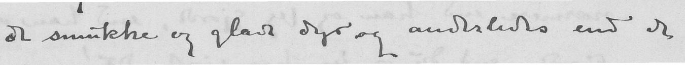de smukke og glade dyr og anderledes end de
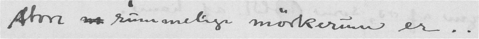store m rummelige mørkerum er..
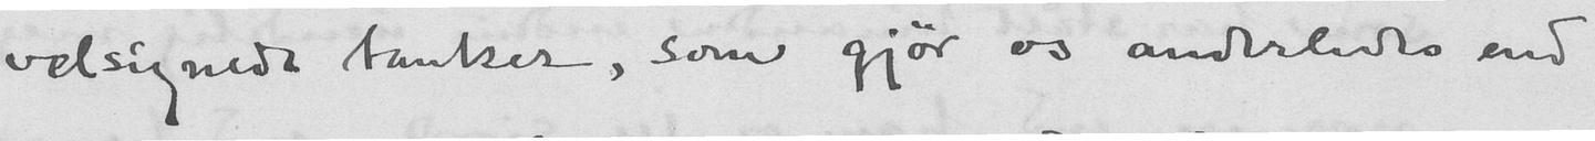velsignede tanker, som gjør os anderledes end
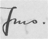Jens.
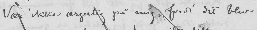Vær ikke ærgerlig på mig, fordi det blev
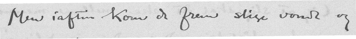Men iaften kom de frem slige vonde og
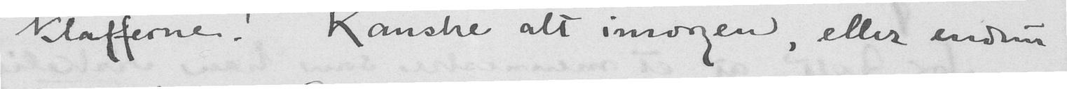klafferne! Kanske alt imorgen, eller endnu
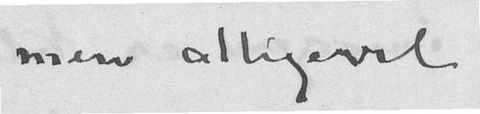men alligevel
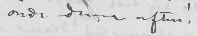onde denne aften!
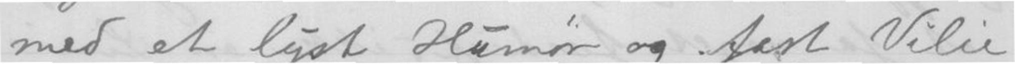med et lyst Humør og fast Vilie
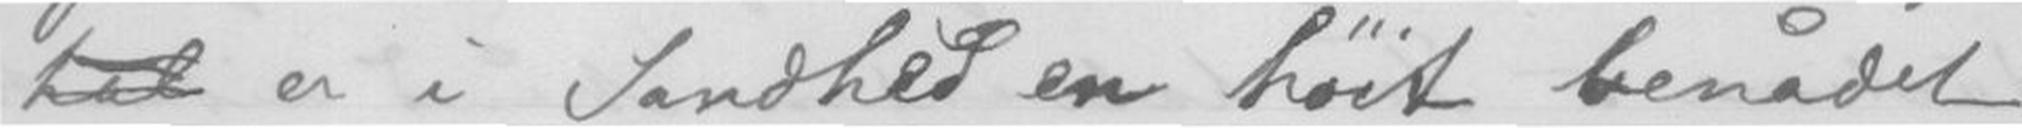hal er i Sandhed en høit benådet
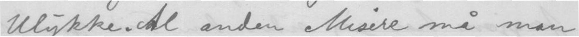Ulykke. Al anden Misere må man
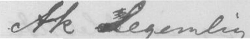Mand. - - - Ak Legemlig
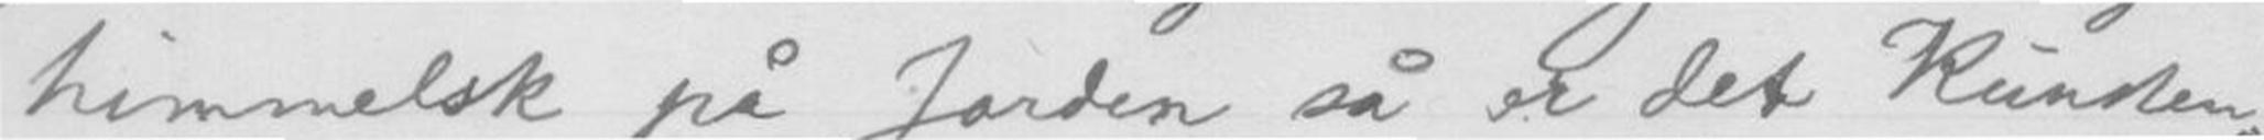himmelsk på Jorden så er det Kunsten,
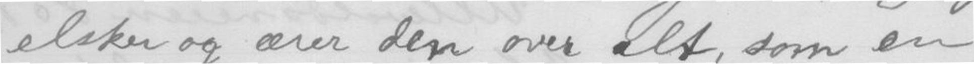elsker og ærer den over alt, som en
